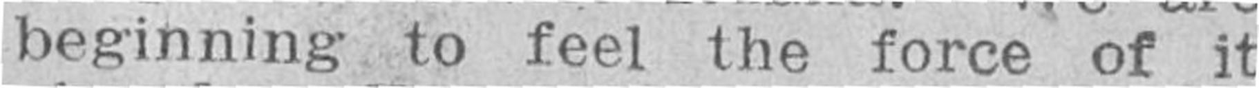beginning to feel the force of it
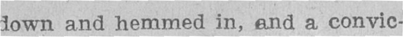lown and hemmed in, and a convic-
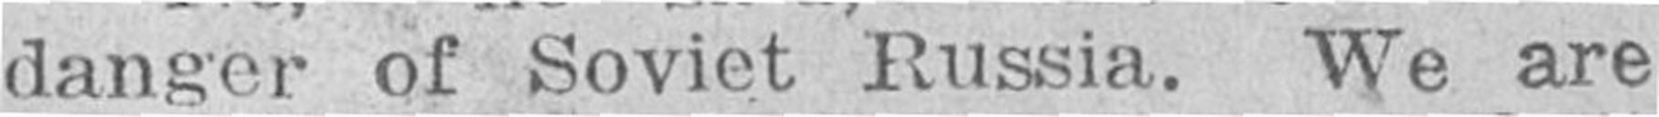danger of Soviet Russia. We are
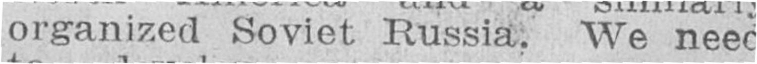organized Soviet Russia. We need
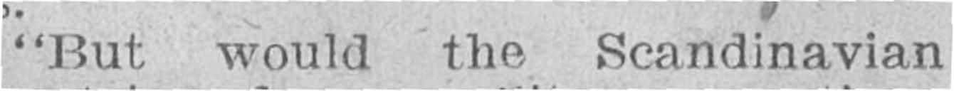"But would the Scandinavian
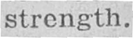strength."
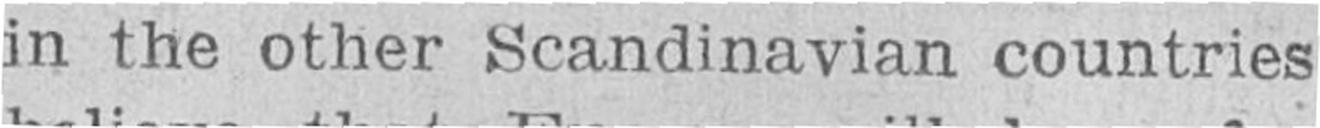in the other Scandinavian countries
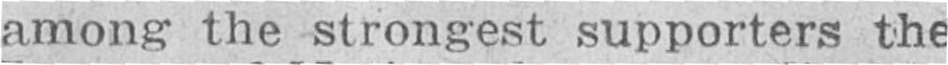among the strongest supporters the
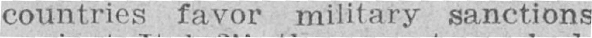countries favor military sanctions
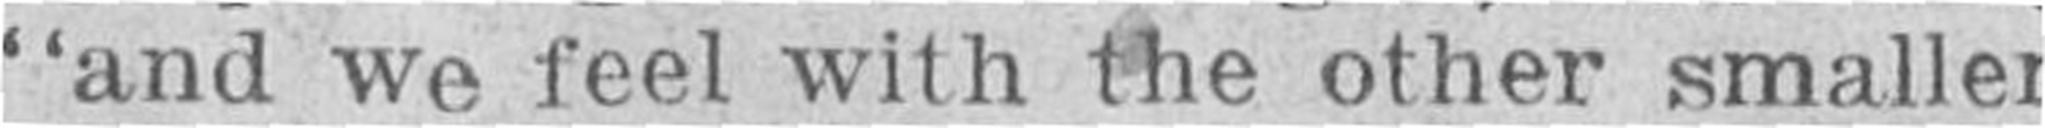and we feel with the other smaller
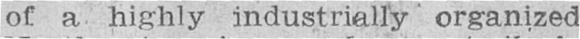of a highly industrially organized
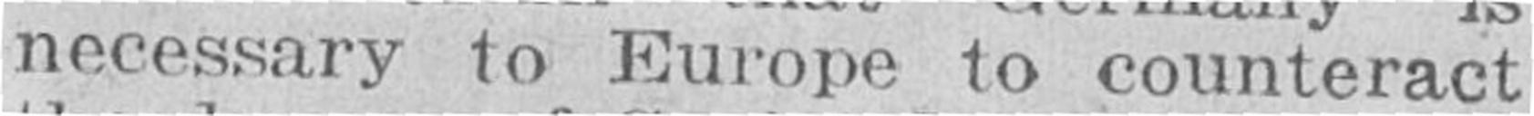necessary to Europe to counteract
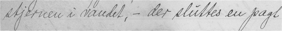stjernen i vandet, - der sluttes en pagt
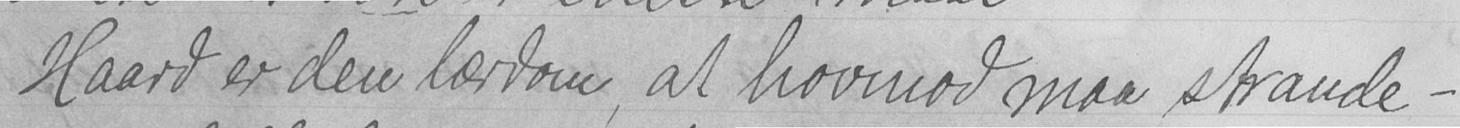Haard er den lærdom, at hovmod maa strande -
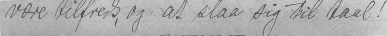være tilfreds, og at slaa sig til taal!
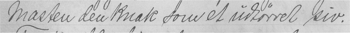Masten den knak som et udtørret siv.
